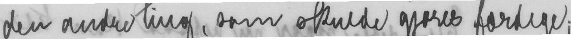den andre ting, som skulde gjøres færdige;
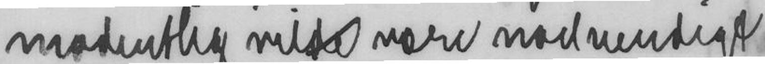modentlig vilde være nødvendigt
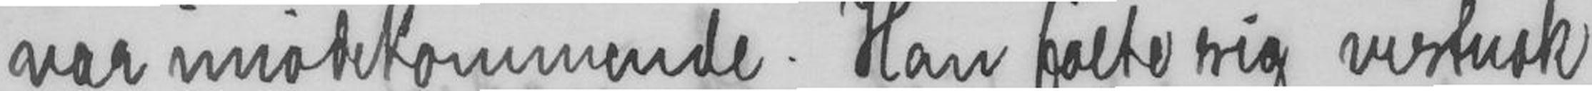var imødekommende. Han følte sig vistnok
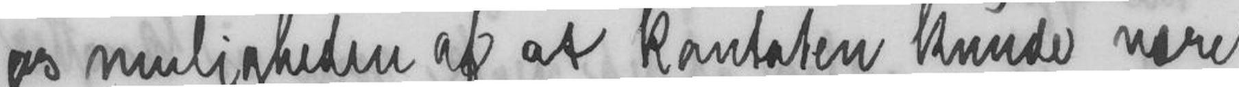os muligheden af at kantaten kunde være
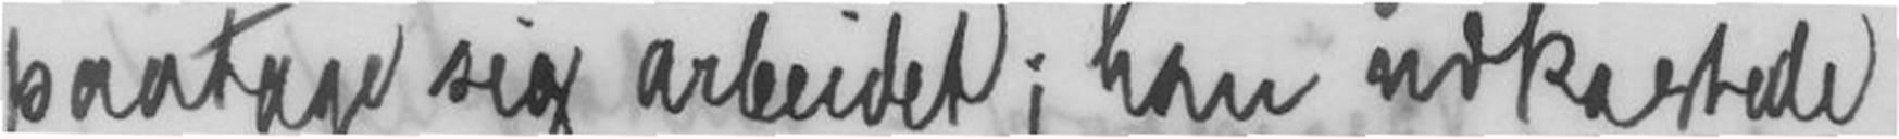paatage sig arbeidet; han udkastede
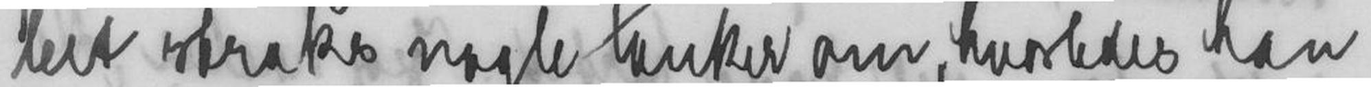bet straks nogle tanker om, hvorledes han
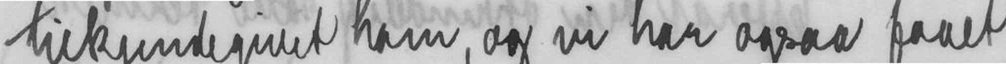tilkjendegivet ham, og vi har ogsaa faaet
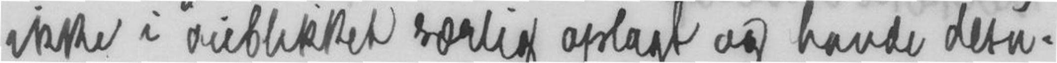ikke i øieblikket særlig oplagt og havde desu-
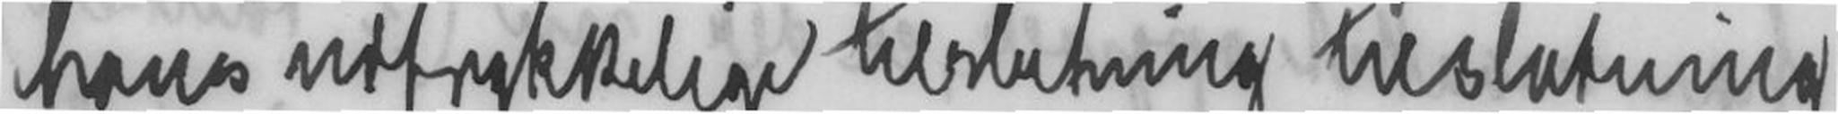hans udtrykkelige tilslutning tilslutning
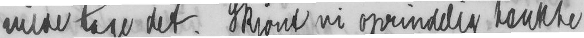vilde tage det. Skjønt vi oprindellig tænkte
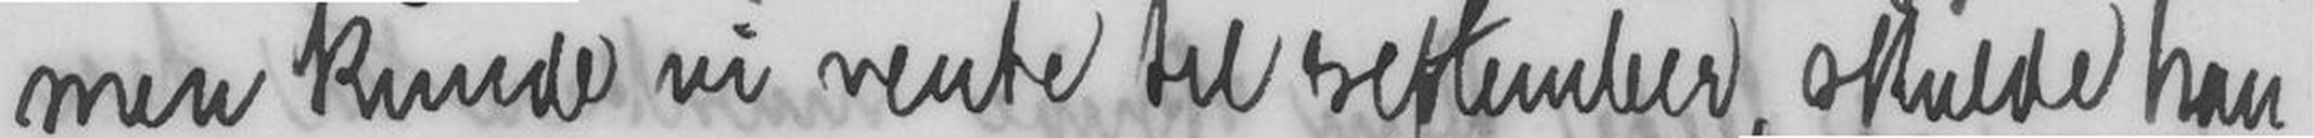men kunde vi vente til september, skulde han
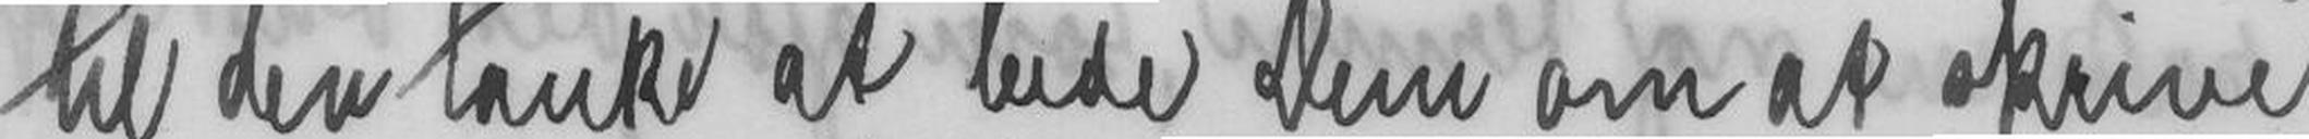til den tanke at bede Dem om at skrive
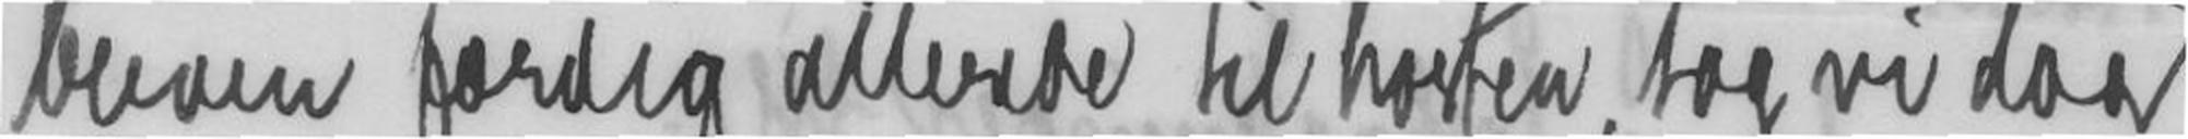bleven færdig allerede til høsten, tog vi dog
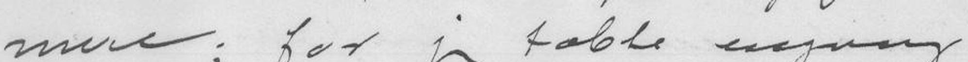mere; for jeg tabte engang
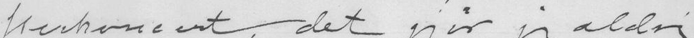sterkoncert, det gjør jeg aldrig
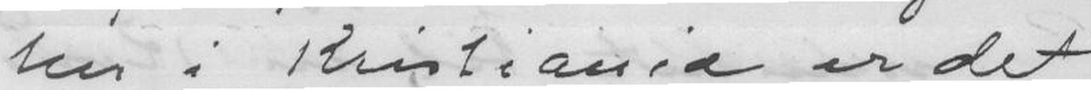her i Kristiania er det
